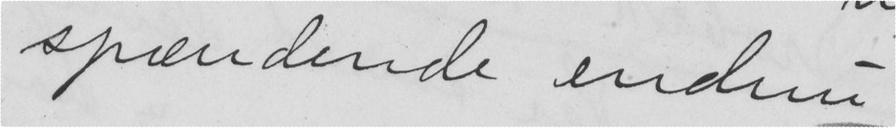spændende endnu
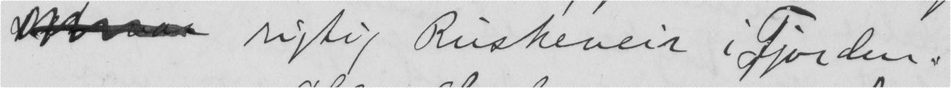rigtig Ruskeveir i Fjorden.
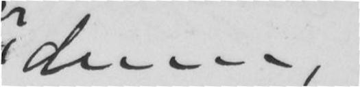dum,
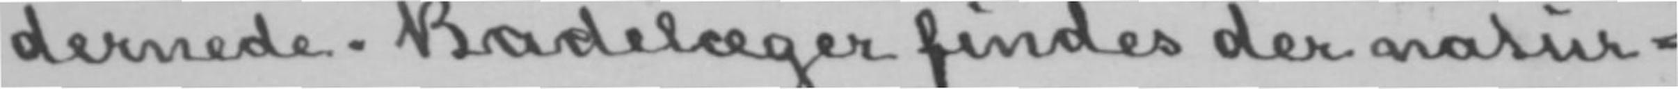dernede. Badelæger findes der natur-
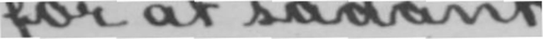for at sådant
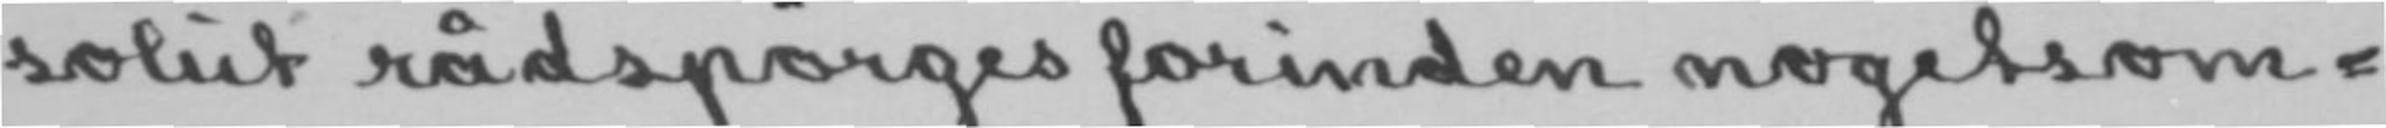solut rådspørges forinden nogetsom-
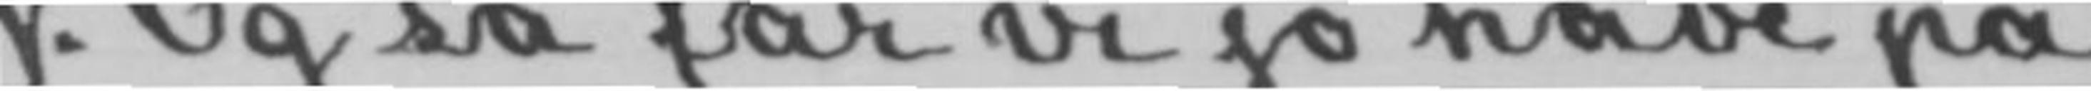Og så får vi jo håbe på
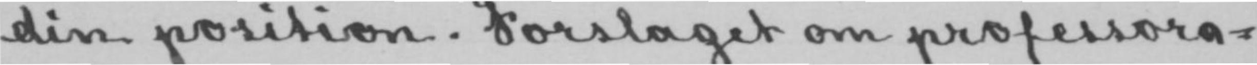din position. Forslaget om professora-
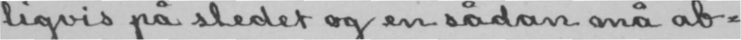ligvis på stedet og en sådan må ab-
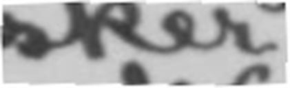sker.
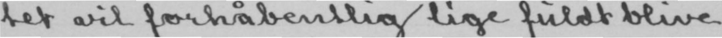tet vil forhåbentlig lige fuldt blive
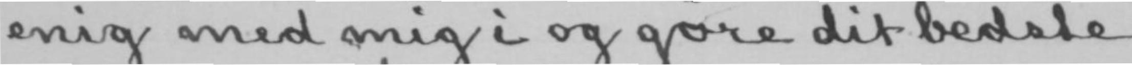enig med mig i og gøre dit bedste
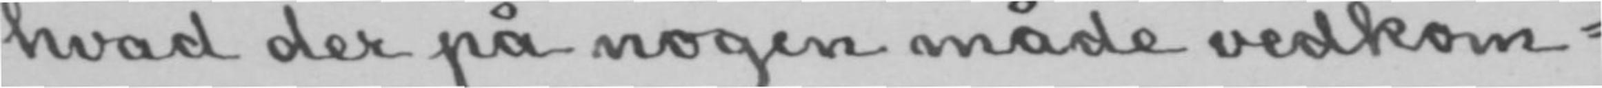hvad der på nogen måde vedkom-
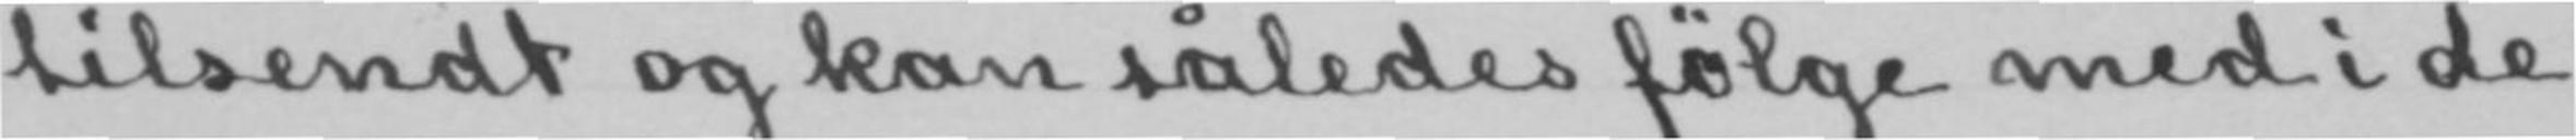tilsendt og kan således følge med i de
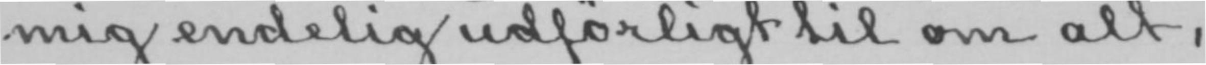mig endelig udførligt til om alt,
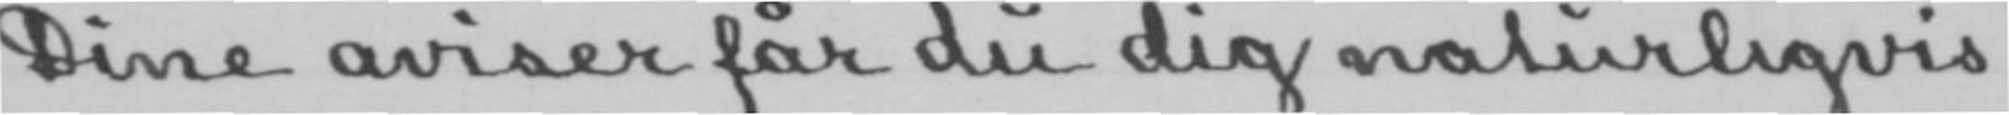Dine aviser får du dig naturligvis
